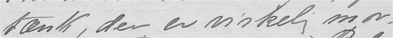tænk, den er virkelig mor-
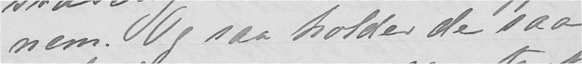nem. Og saa holder de saa
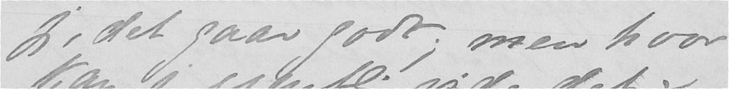jeg, det gaar godt; men hvor
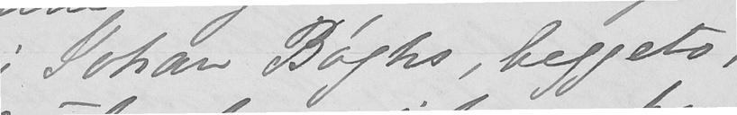i Johan Bøghs, beggeto,
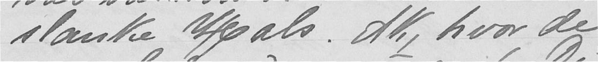slanke Hals. Ak, hvor de
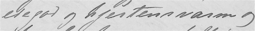eiegod og hjertensvarm og
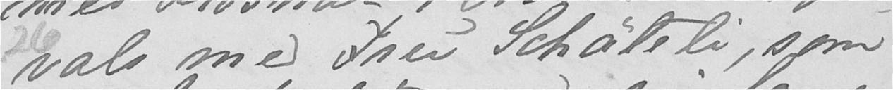vals med Fru Schäteli, som
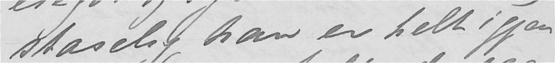staselig han er helt igjen-
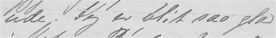ude. Jeg er blit saa glad
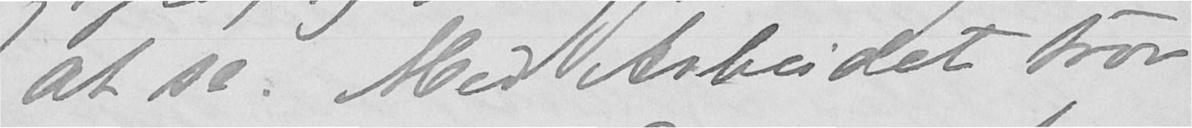at se. Med Arbeidet tror
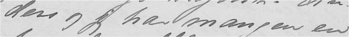ders og jeg har mangen en
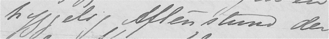hyggelig Aftenstund der
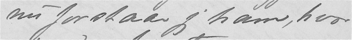nu forstaar jeg ham, hvor
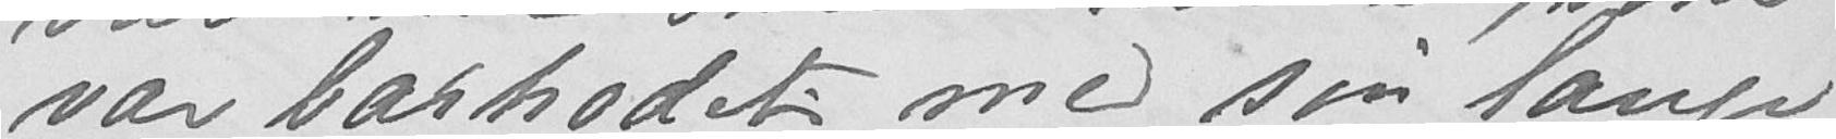var barhodet med sin lange
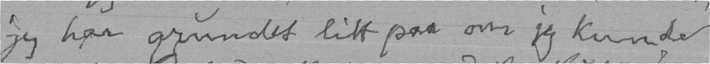jeg har grundet litt paa om jeg kunde
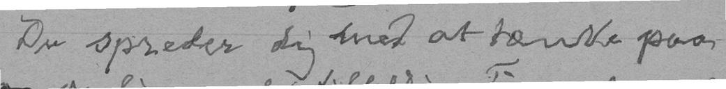Du spreder dig med at tænke paa
